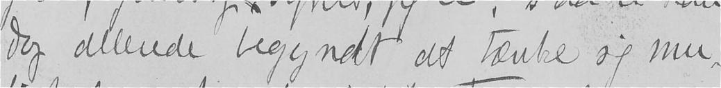og allerede begyndt at tænke sig mu-
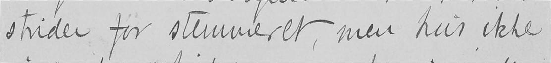strider for stemmeret, men hvis ikke
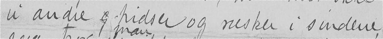vi andre og hidser og resker i sindene,
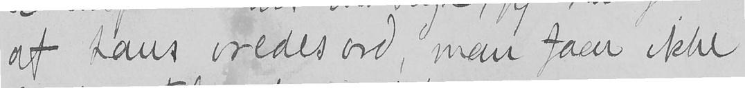af hans vredes ord, man faar ikke
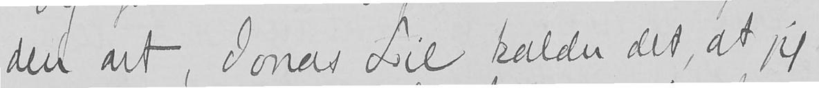den art, Jonas Lie kalder det, at jeg
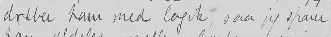dræber ham med logik, saa jeg sparer
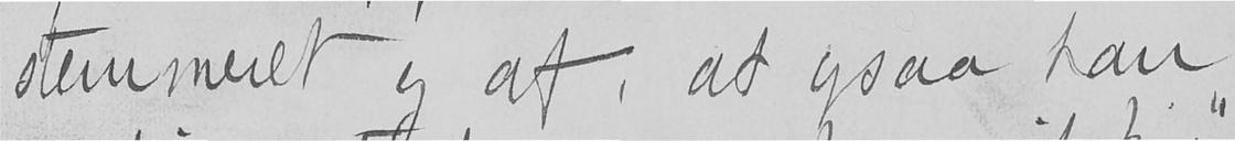stemmeret og af, at ogsaa han
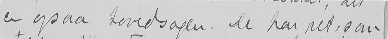er ogsaa hovedsagen. De har ret, som
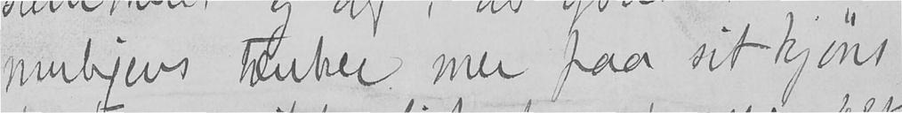muligens tænker mer paa sit kjøns
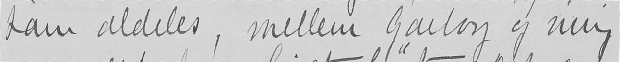ham aldeles, mellem Garborg og mig
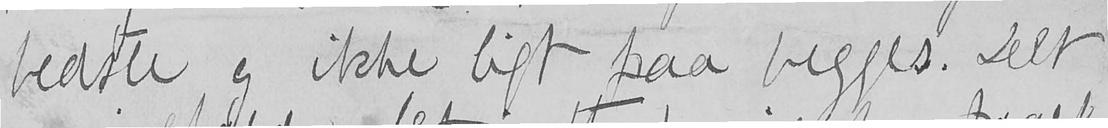bedste og ikke ligt paa begges. Det
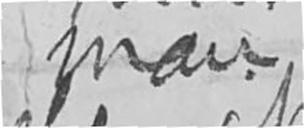man
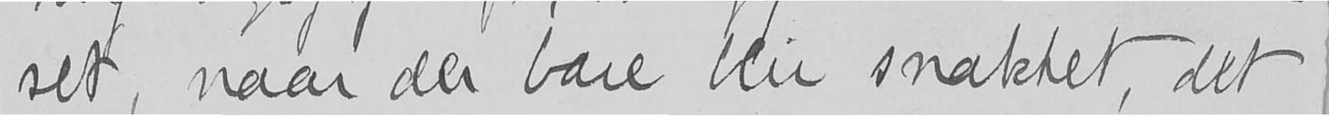set, naar der bare blir snakket, det
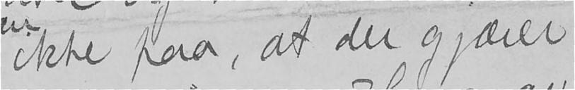ikke paa, at der gjærer
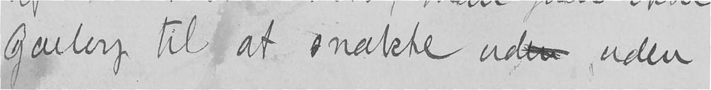Garborg til at snakke uden uden
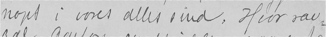noget i vores alles sind. Hvor rav-
